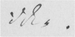ikke.
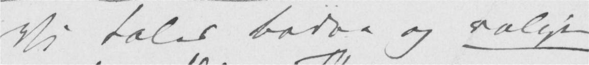Vi taler bedre og roligere
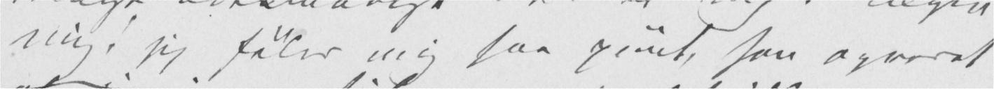mig! jeg føler mig saa piint, saa oprevet
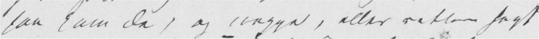saa kom De, og neppe, eller rettere sagt
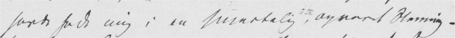havde sadt mig i en smertelig, oprevet Stemning –
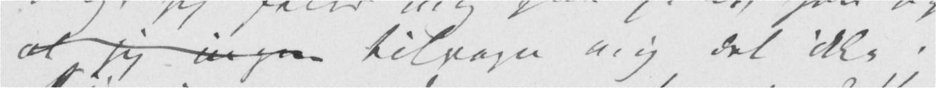at jeg ingen tilregn mig det ikke.
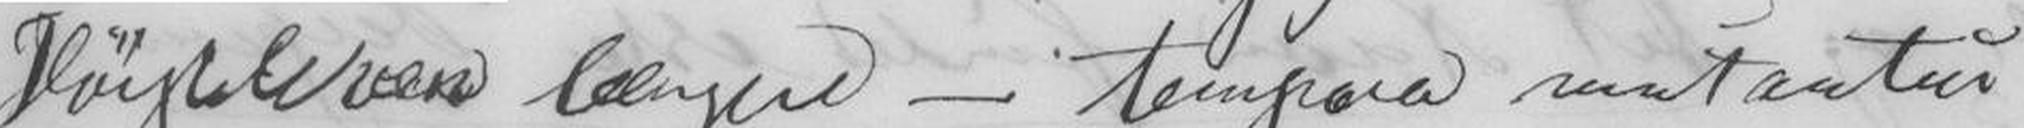Høiskoleven længere - tempora mutantur,
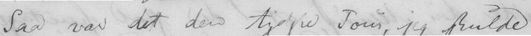Saa var det den tydske Tour, jeg skulde
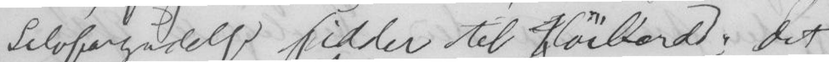Selvforgudelse sidder til Høibord; det
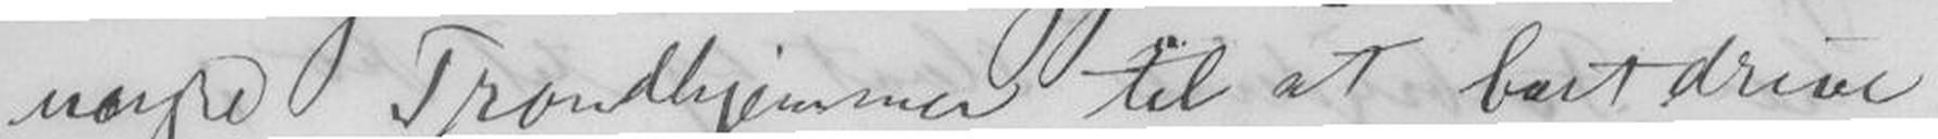norske Trondhjemmer til at bortdrive
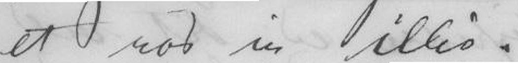et nos in illes.
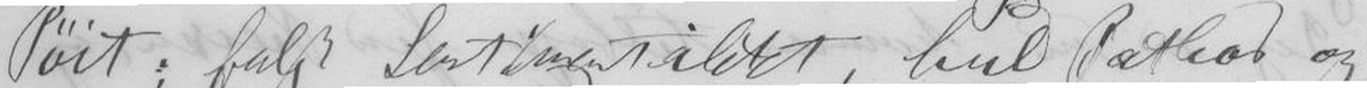Pøit; falsk Sentimentalitet, hul Pathos og
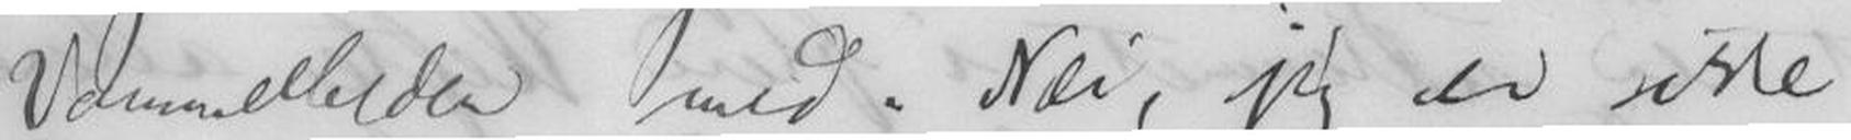Vammelheden med. Nei, jeg er ikke
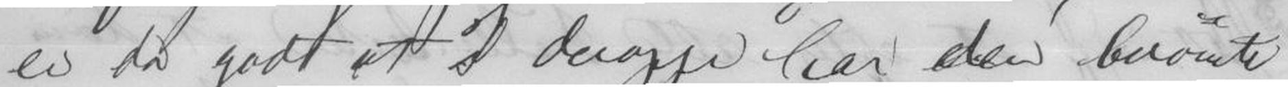er da godt at I deroppe har den berømte
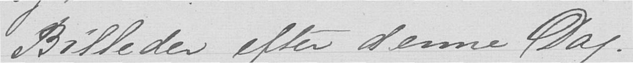Billeder efter denne Dag.
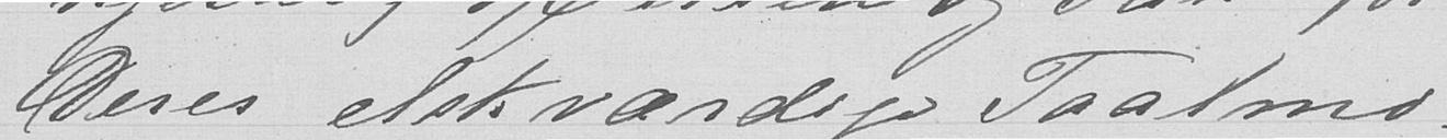Deres elskværdige Taalmo-
